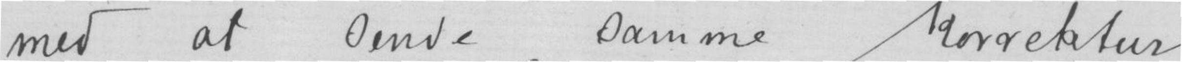med at sende samme korrektur
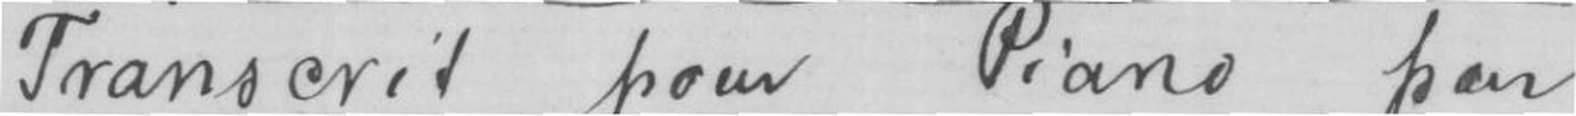Transcrit pom Piano par
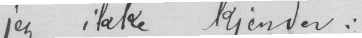jeg ikke kjender:
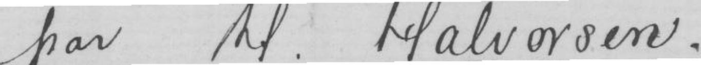par st. Halvorsen.
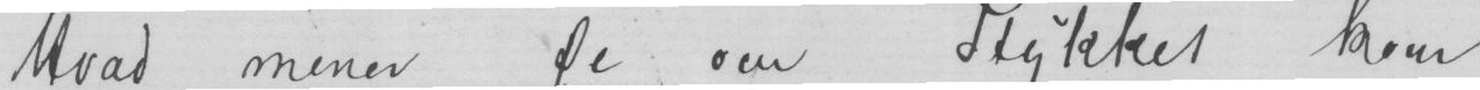Hvad mener De som Stykket kom
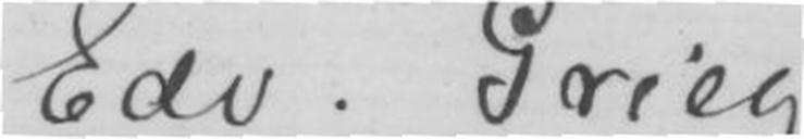Edv. Grieg
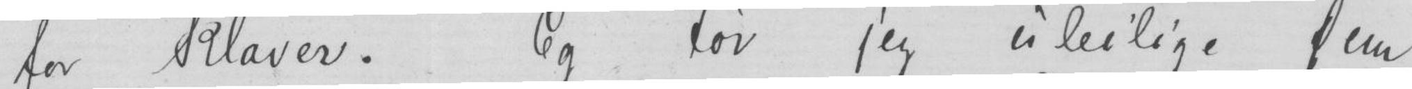for klaver. Og tør jeg uleilige Dem'
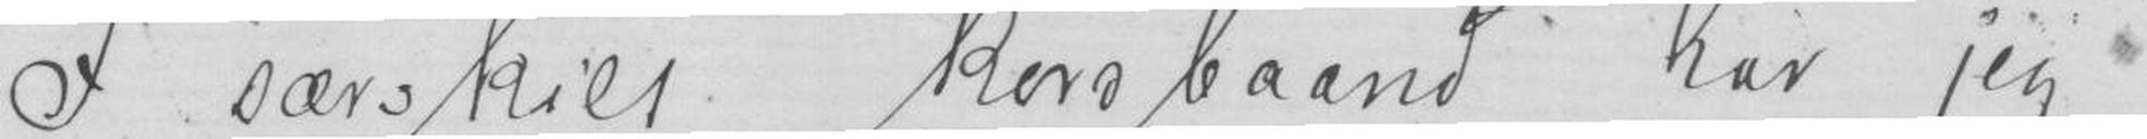I særskill korsbaand har jeg
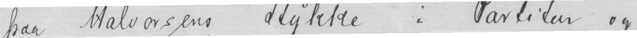paa Halvorsens Stykke i Partiten og
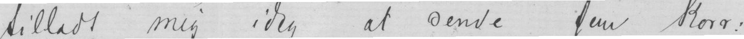tilladt mig idag at sende Dem korr.
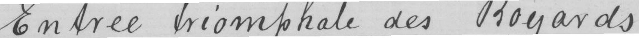Entrèe triomplhale des Boyards
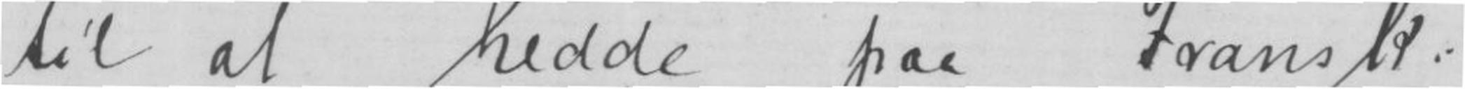til at hedde paa Fransk.
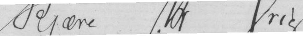Kjære Hr. Grieg!
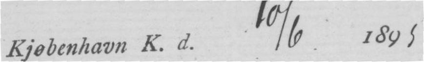Kjøbenhavn K.d. 10/6 1895
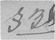§ 38
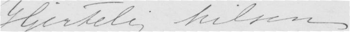Hjertelig hilsen
